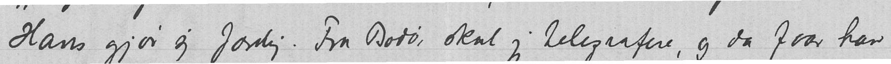Hans gjør sig færdig. Fra Bodø skal jeg telegrafere, og da faar han
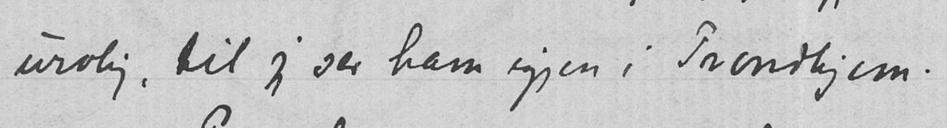urolig, til jeg ser ham igjen i Trondhjem.
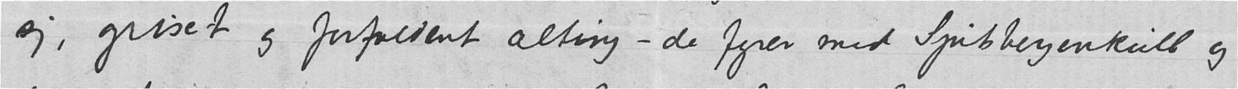sig, griset og forfaldent alting – de fyrer med Spitsbergenkull og
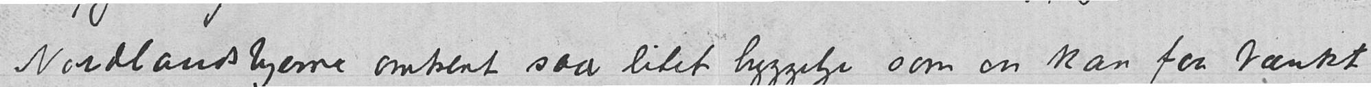Nordlandsbyerne omtrent saa litet hyggelige som en kan faa tænkt
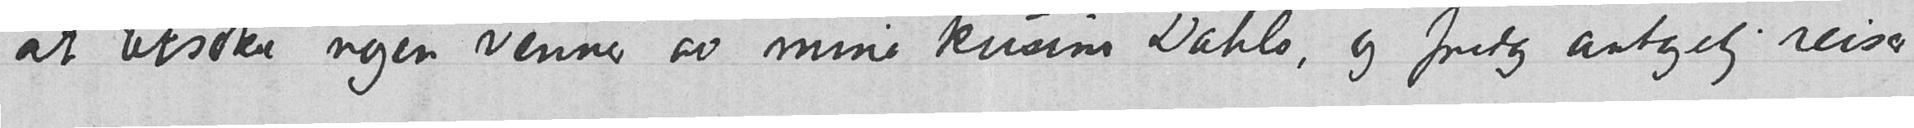at besøke nogen venner av mine kusiner Dahls, og fredag antagelig reiser
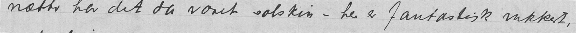nætter har det da været solskin – her er fantastisk vakkert,
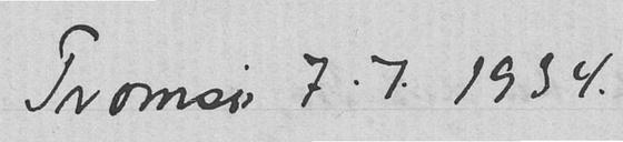Tromsø 7.7.1934.
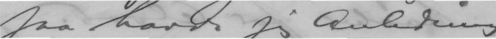og saa havde jeg Anledning
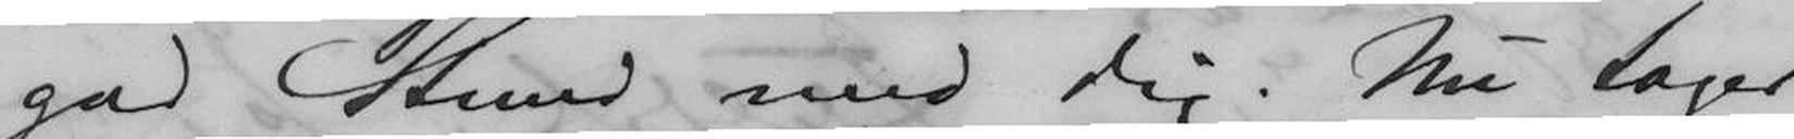god Stund med Dig. Nu tager
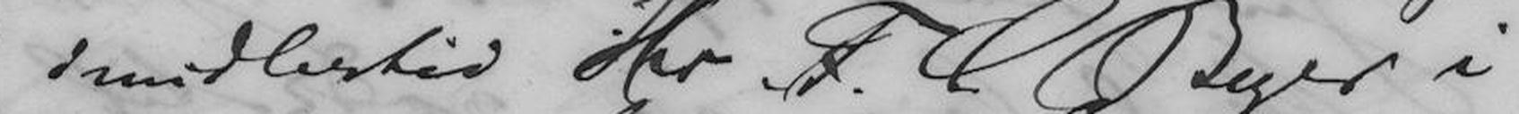jeg imidlertid Hr F.C. Beyer i
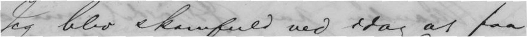Jeg blev skamfuld ved idag at faa
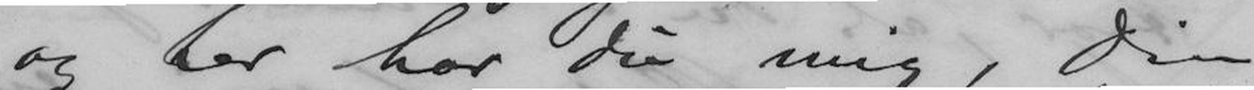og her har du mig, Din
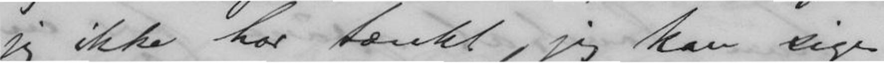jeg ikke har tænkt, jeg kan sige
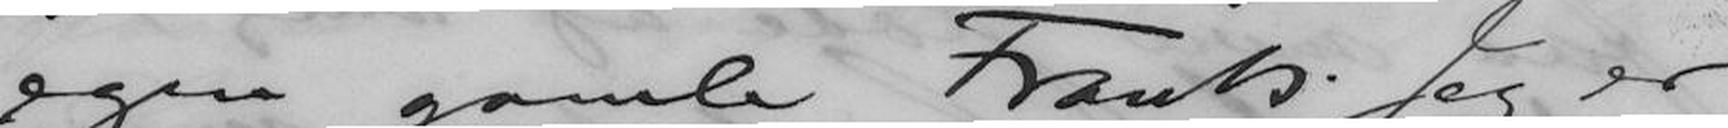egen gamle Frants. Jeg er
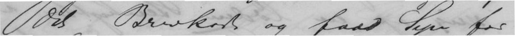det Brevkort og faad Syn for
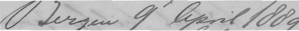Bergen 9de April 1889.
